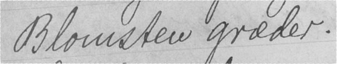Blomsten græder.
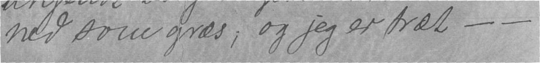ned som græs; og jeg er træt - -
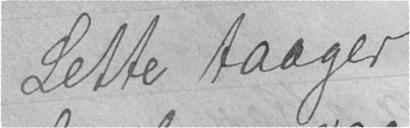Lette taager
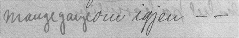mange gange om igjen - -
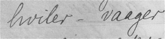hviler - vaager
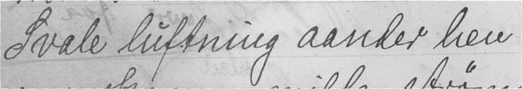Svale luftning aander hen
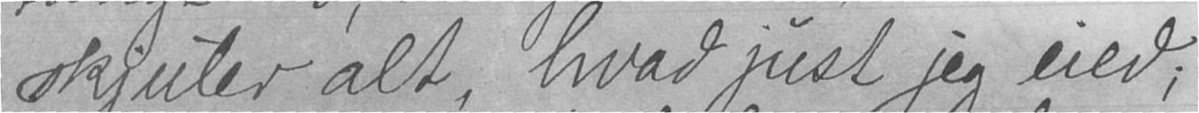skjuler alt, hvad just jeg eied;
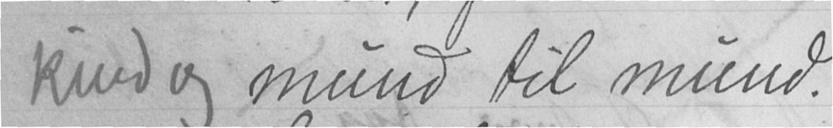kind og mund til mund.
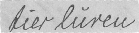tier luren
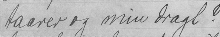taarer og min dragt?
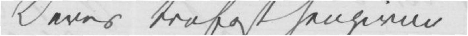Deres trofast hengivne
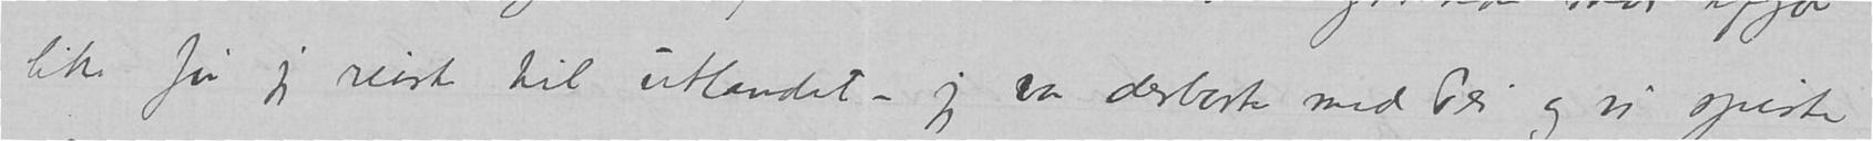like før jeg reiste til utlandet – jeg var derborte med Pei og vi spiste
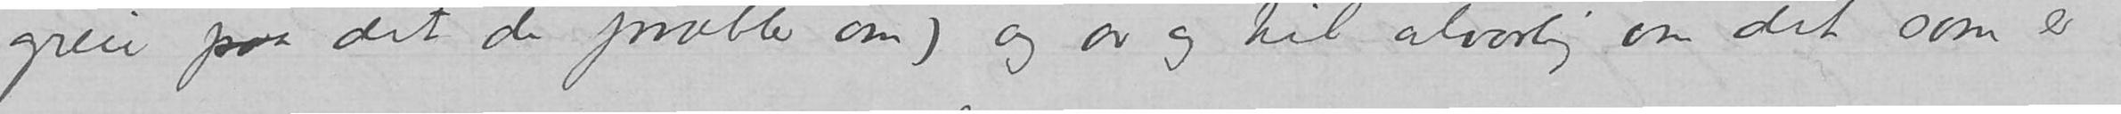greie paa det de prabler om) og av og til alvorlig om det som er
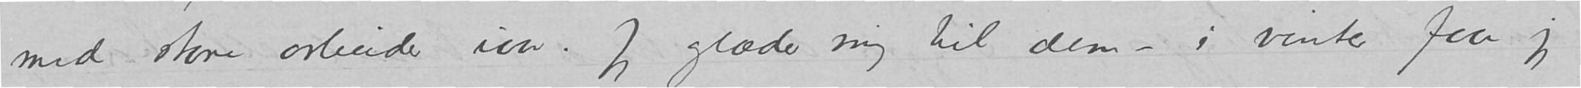med store arbeider iaar. Jeg glæder mig til dem – i vinter faar jeg
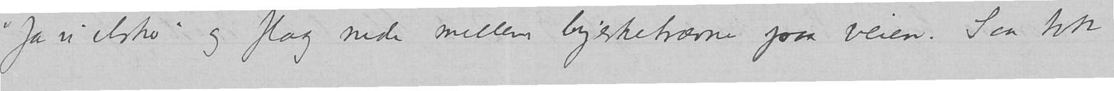«Ja vi elsker» og flag nede mellem hjerketrærne paa veien. Saa tok
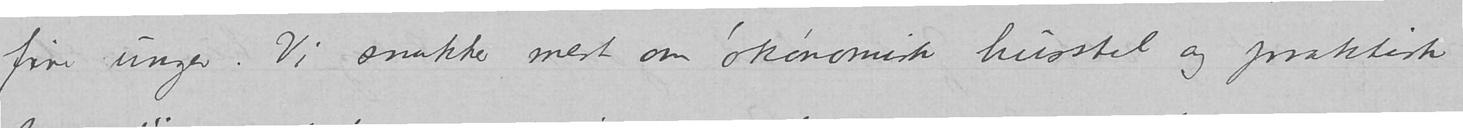fire unger. Vi snakker, mest om økonomisk husstel og praktisk
